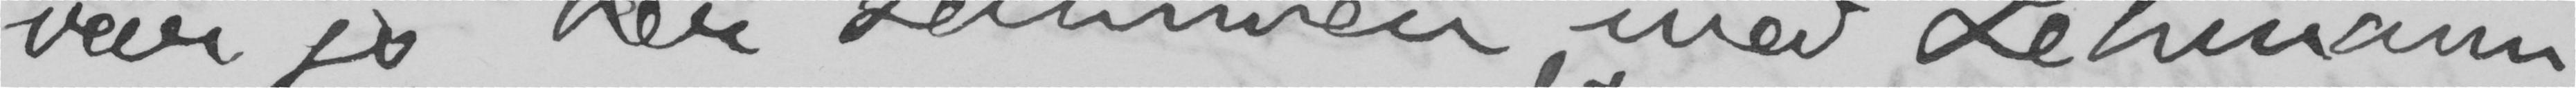var jo den sommeren med Lehmann
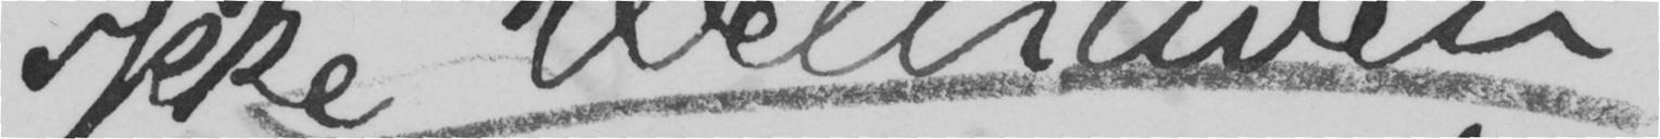ikke Welhaven:
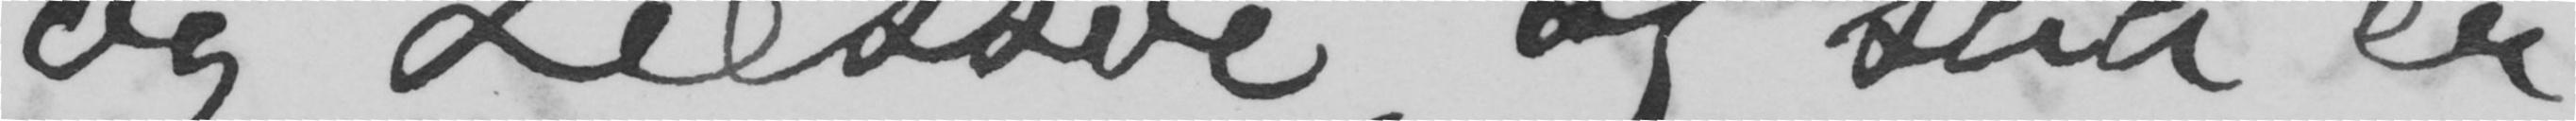og Lessøe, og saa er
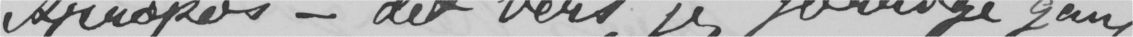Apropos – det vers, jeg forrige gang
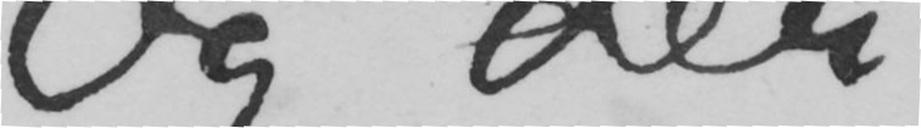Og der
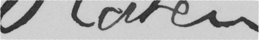Platen.
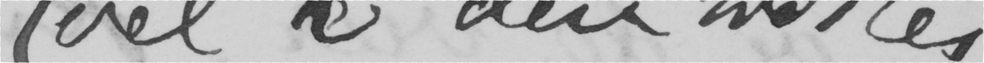vel i den sidstes
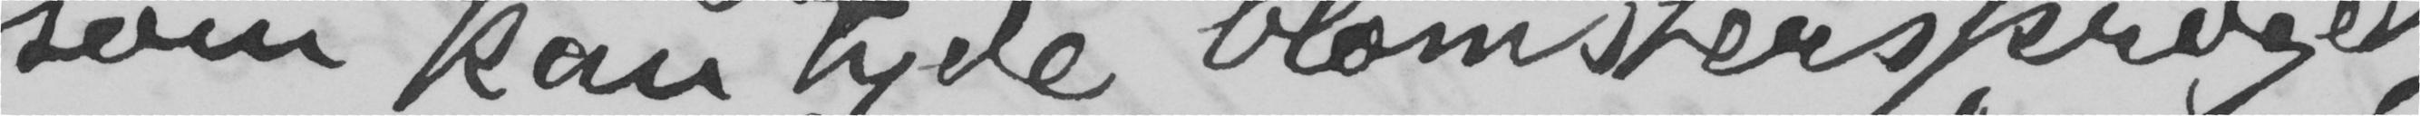som kan tyde blomstersproget,
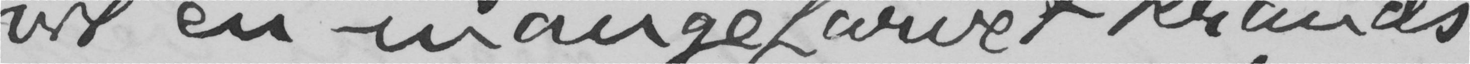vil en mangefarvet Krands
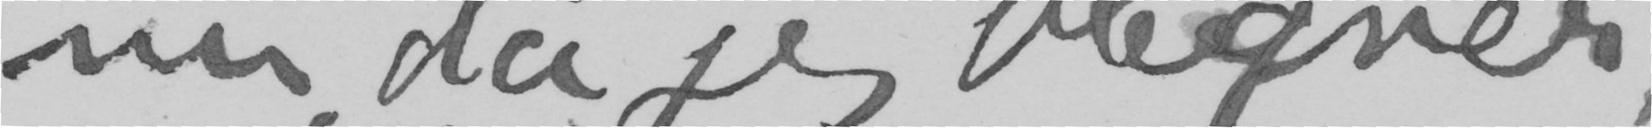nu, da jeg blegner
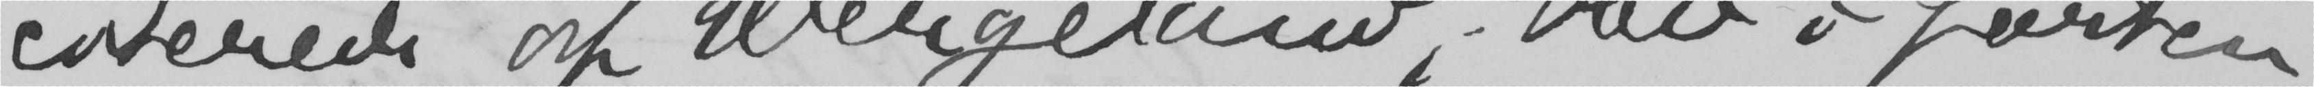citerede af Wergeland, blev i farten
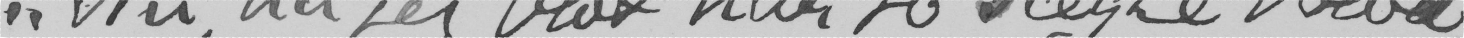«Nu, da jeg blot har to Pægle Blod,
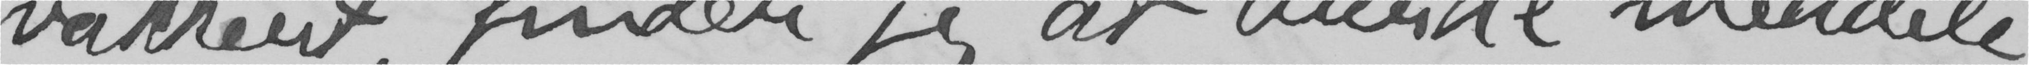vakkert, finder jeg at burde meddele
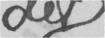det
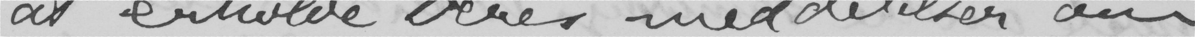at erholde Deres meddelelser om
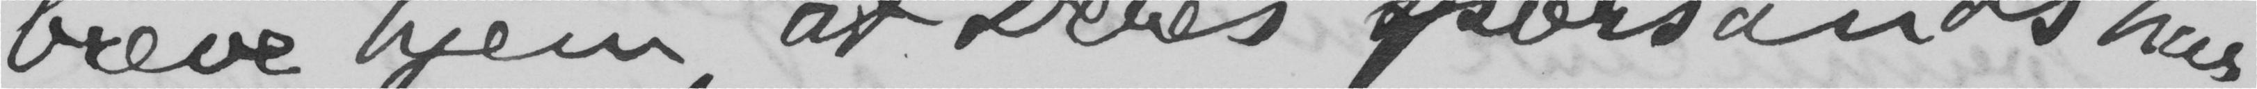breve hjem, at Deres sporsands har
